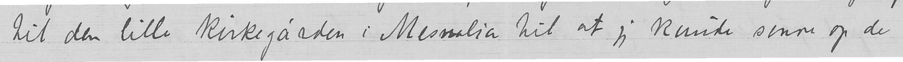til den lille kirkegården i Mesnalia til at jeg kunde sønne op de
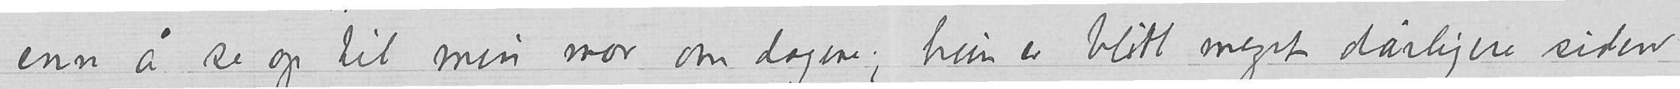enn av se op til min mor om dagene; hun er blitt meget dårligere siden
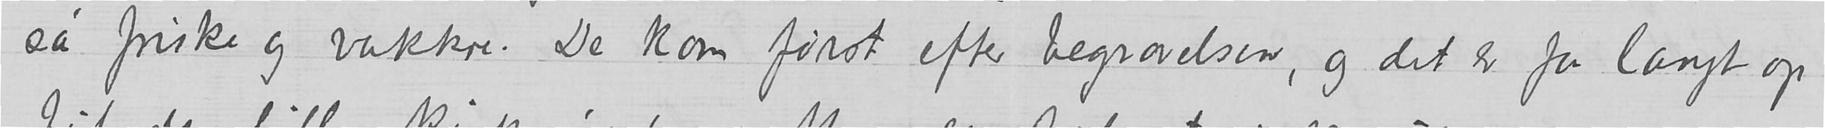så friske og vakkre. De kom først efter begravelsen, og det er for langt op
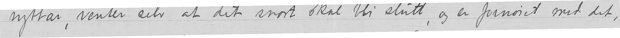nyttår, venter selv at det snart skal bli slutt, og en fornøiet med det,
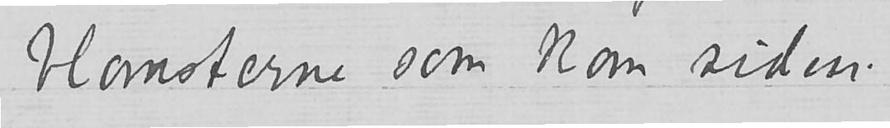blomsterne som kom siden
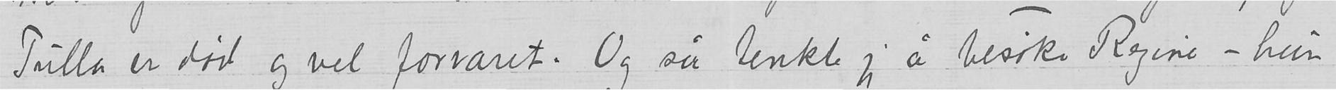Tulla er død og vel forvaret. Og sa tenkte jeg å besøke Regine – hun
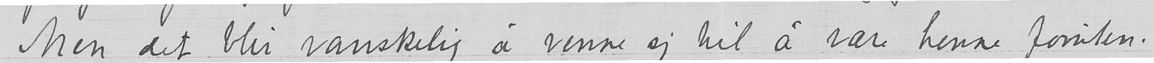Men det blir vanskelig av venne sig til å være henne foruten.
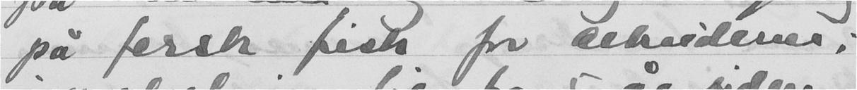på fersk fisk for arbeiderne;
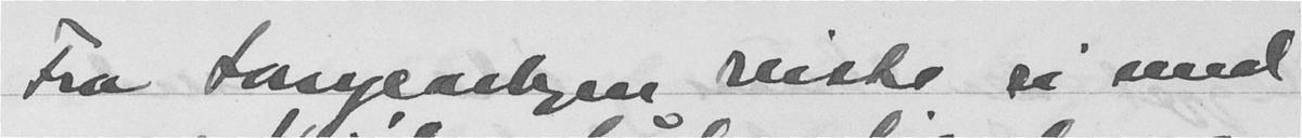Fra Longyearbyen reiste vi med
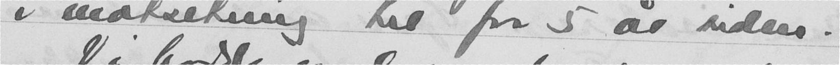i motsetning til for 5 år siden.
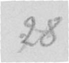28
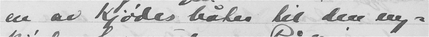en av Kjødes båter til den ny-
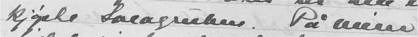kjøpte Sveagruben. På veien
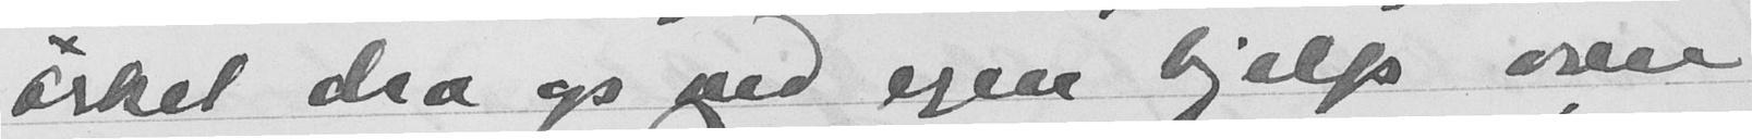orket dra op ved egen hjælp over
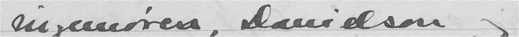ingeniører, Davidson og
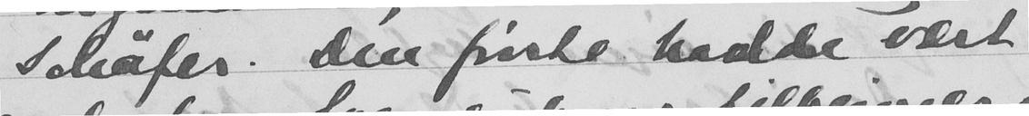Schäfer. Den første havde vært
